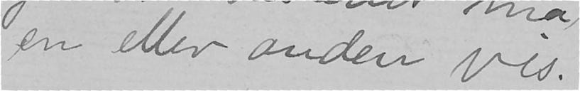en eller anden vis.
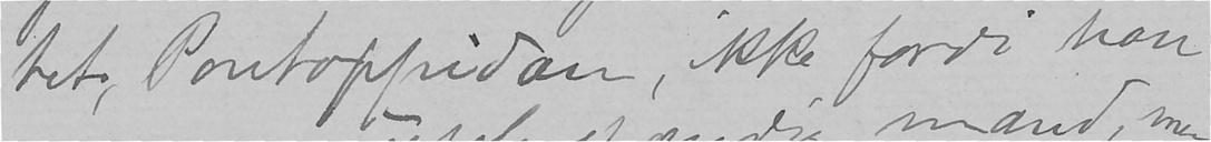tet, Pontoppidan, ikke fordi han
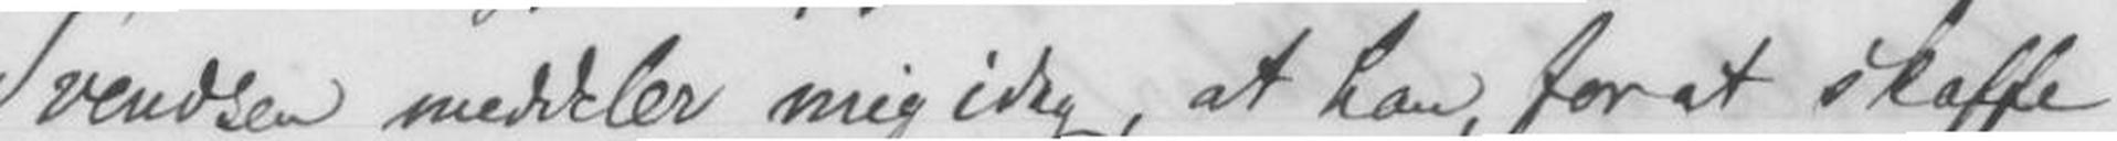Svendsen meddeler mig i dag, at han, for at skaffe
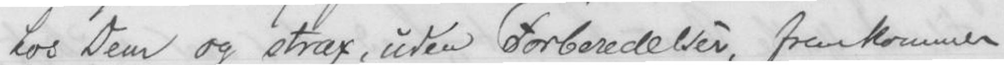hos dem og strax, uden Forberedelser, framkommer
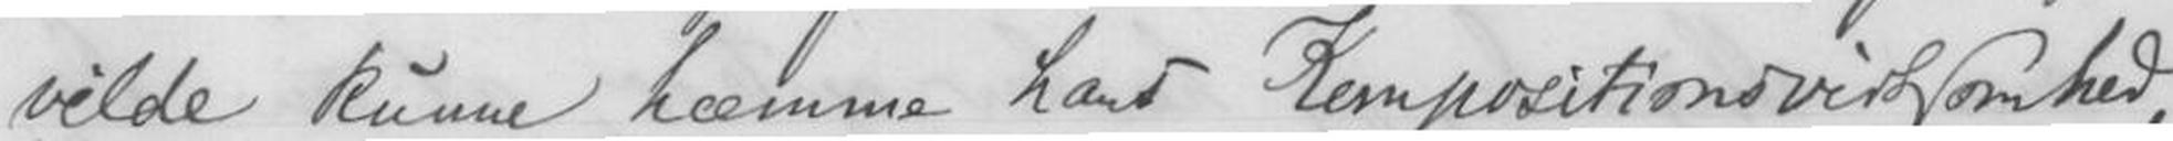vilde kunne komme hans Kompositionsvirksomhed,
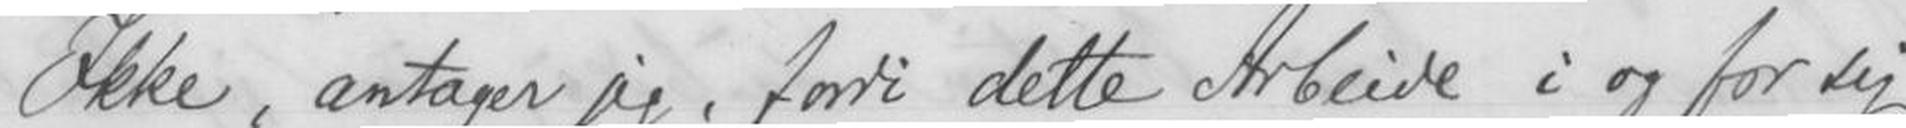Ikke, antager jeg, fordi dette Arbeide i og for sig
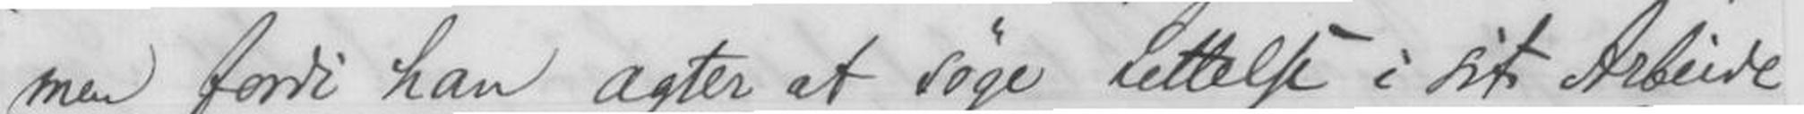fordi han agter at søge lettelse i sit Arbeide
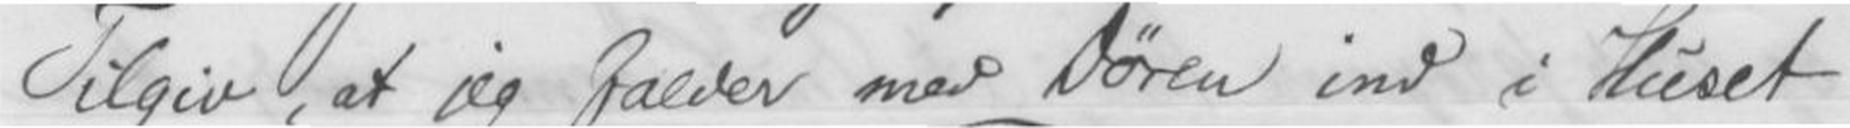Tilgiv, at jeg falder mod Døren ind i Huset
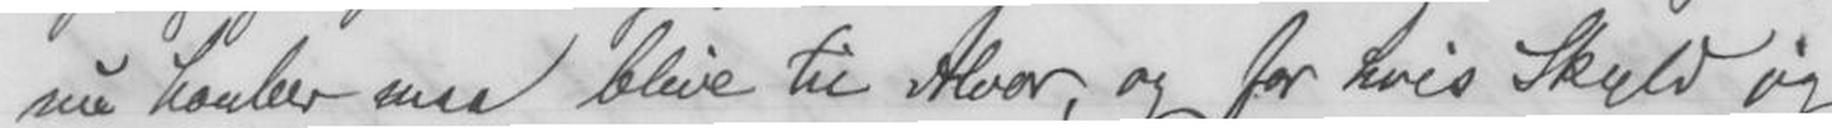nu haaber maa blive til Alvor, og for skyld og
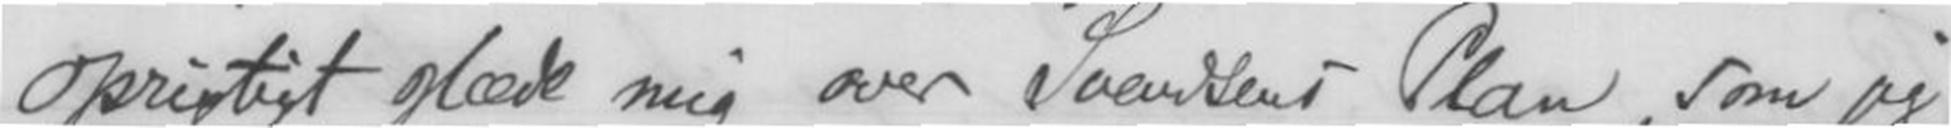oprigtigt glæde mig over Svendsens Plan, som jeg
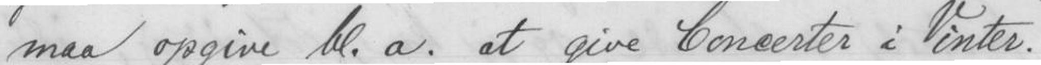maa opgive bl. a. at give Concerter i Vinter.
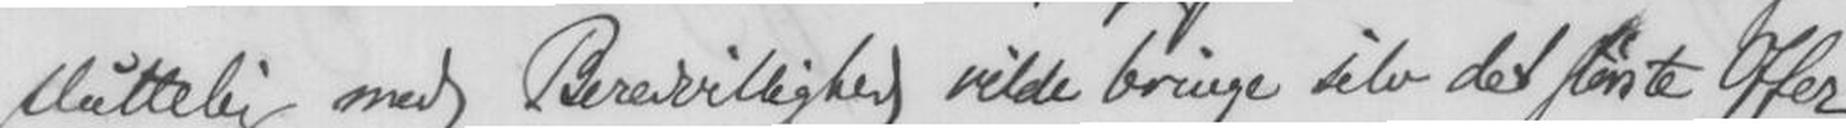med Beredvillighed vilde bringe selv det offer.
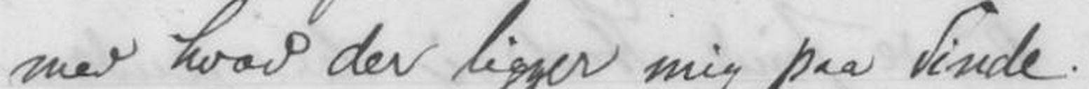med hvad der ligger mig paa Sinde.
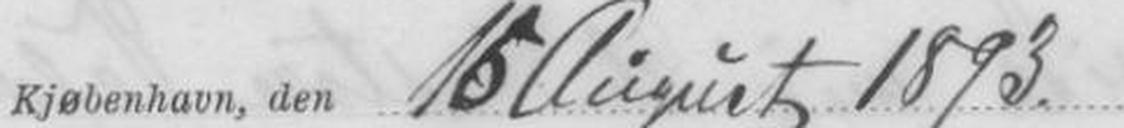Kjøbenhavn, den 16 August 1893.
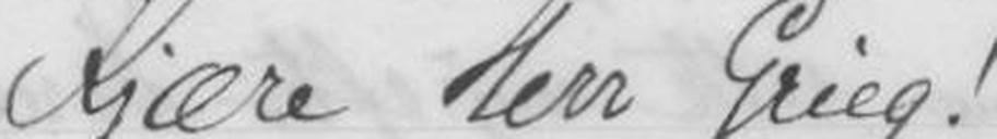Kjære Herr Grieg!
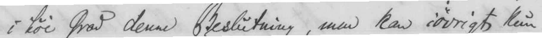i høie Ord denne Beslutning, saa kan i øvrigt kun
